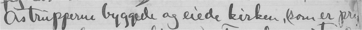Aastrupperne byggede og eiede kirken, (som er pre
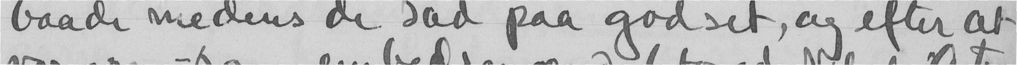baade medens de sad paa godset, og efter at
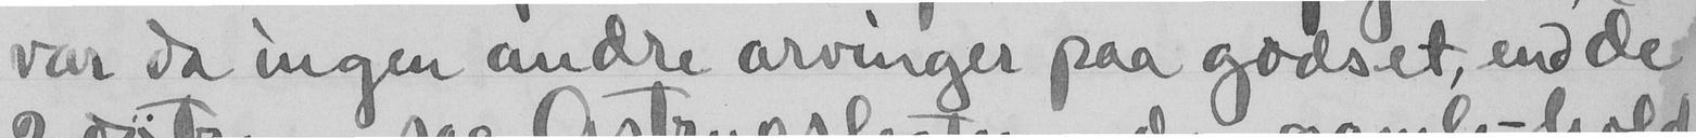var da ingen andre arvinger paa godset, end de
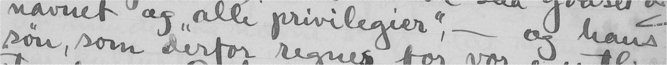navnet og "alle priviligier", - af hans
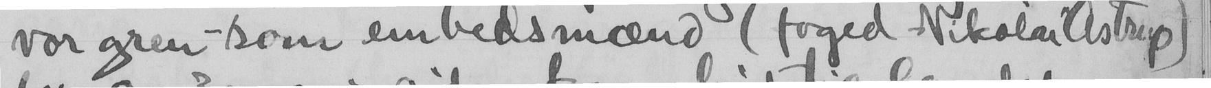vor gren som embedsmænd- (foged Nikolai Astrup)
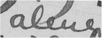alene
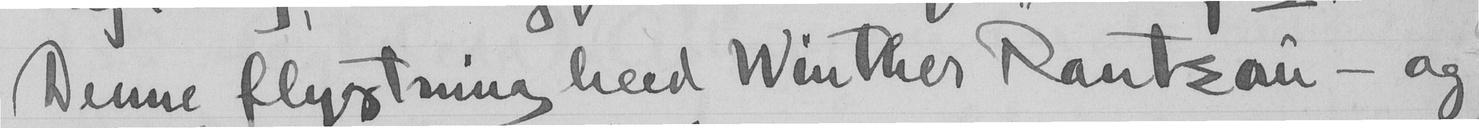Denne flystning heed Winther Rantzau - og
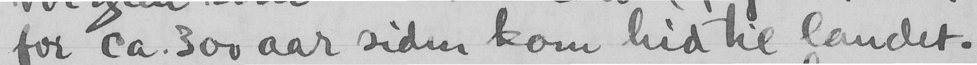for ca. 300 aar siden kom hid til landet.
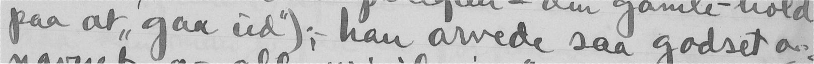paa at "gaa ud"); - han arvede saa godset og
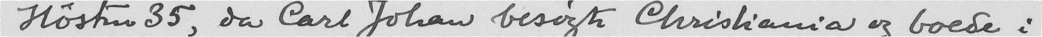Høsten 35, da Carl Johan besøgte Christiania og boede i
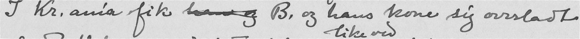I Kr. ania fik han og B. og hans kone sig overladt
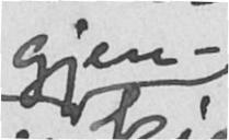gjen-
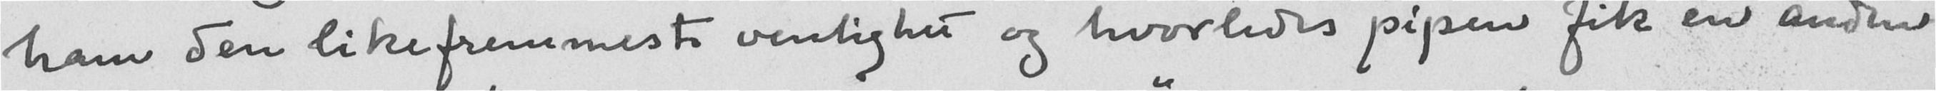ham den likefremmeste venlighet og hvorledes pipen fik en anden
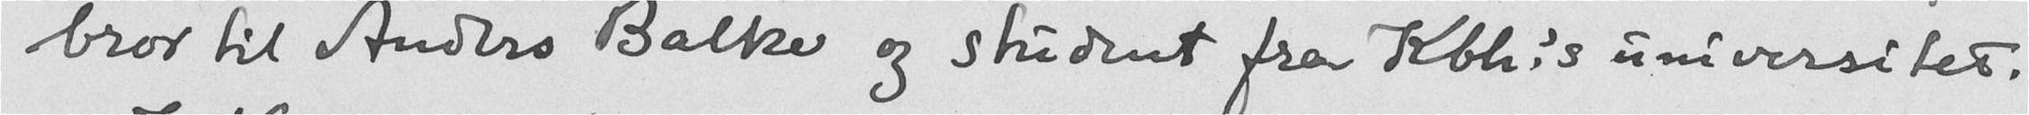bror til Anders Balke og student fra Kbh.'s universitet.
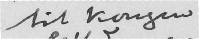til kongen
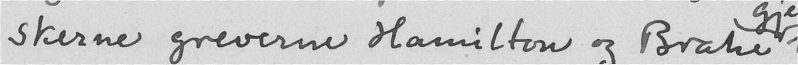skerne greverne Hamilton og Brahe
